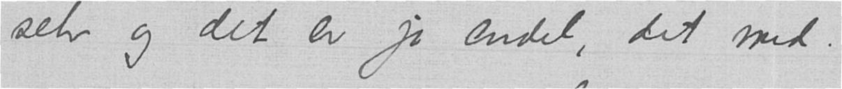selv og det er jo en del, det med.
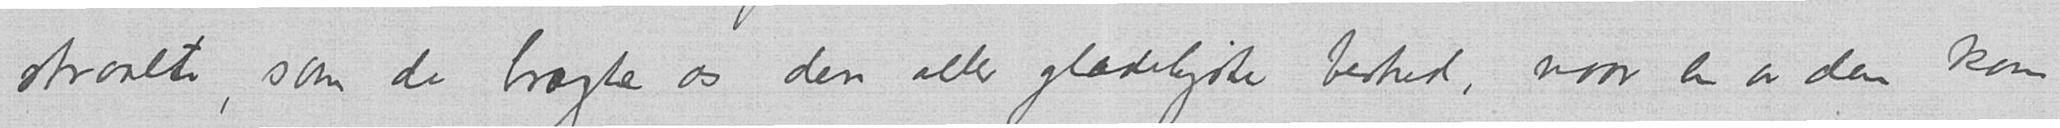straalte, som de bragte os den aller glædeligste besked, naar en av den kom
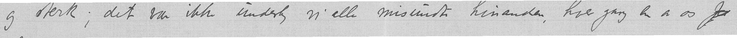og sterk; det var ikke underlig vi alle misundte hinanden, hver gang en av os for
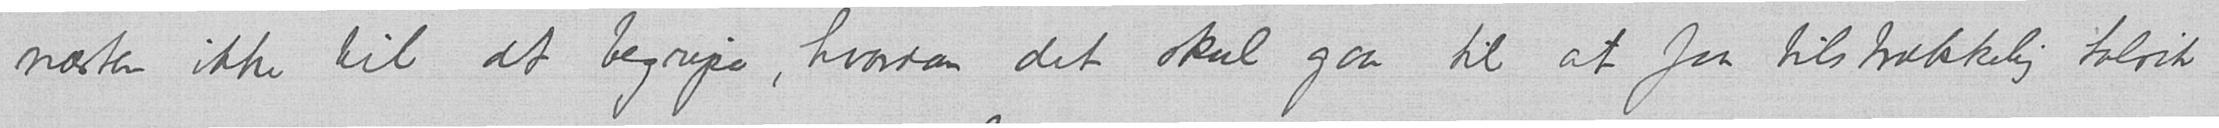næsten ikke til at begripe, hvordan det skal gaa til at faa tilstrækkelig talrik
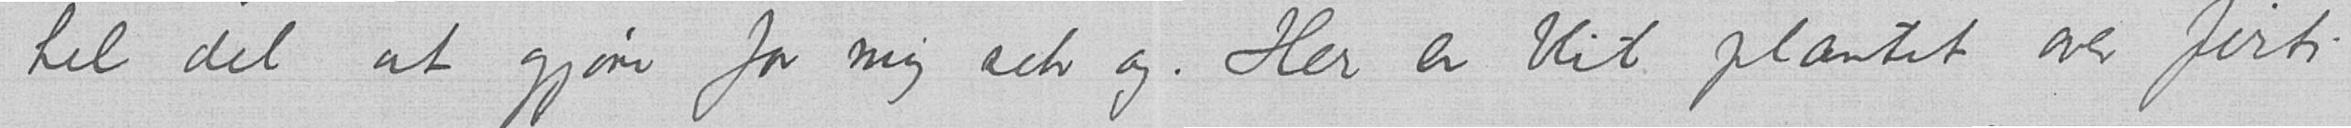hel del at gjøre for mig selv og. Her er blit plantet over firti
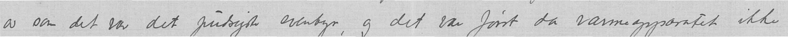av som det var det pudsigste eventyr, og det var først da varmeapparatet ikke
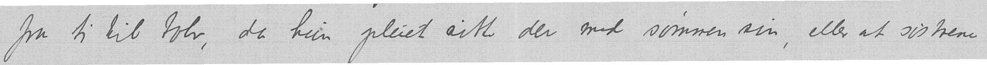fra ti til tolv, da hun pleiet sitte der med sømmen sin, eller at søstrene
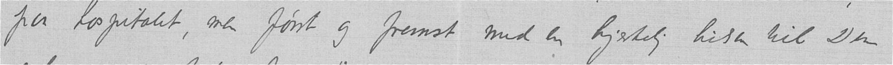paa hospitalet, men først og fremst med en hjertelig hilsen til Dem
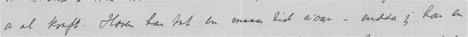av al kraft. Haven har tat en masse tid iaar –- endda jeg har en
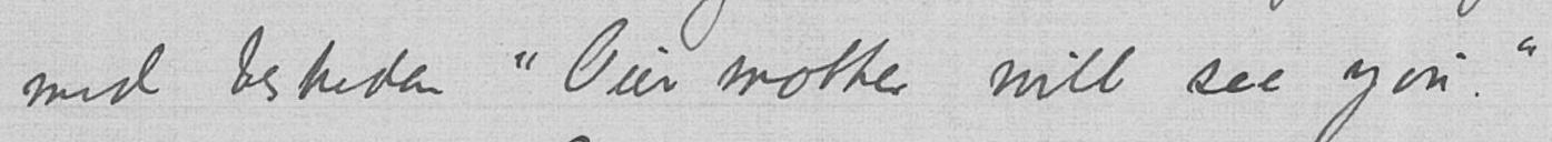med beskeden "Our mother will see you."
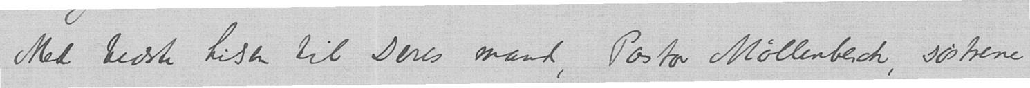Med bedste hilsen til Deres mand, Pastor Møllenbeck, søstrene
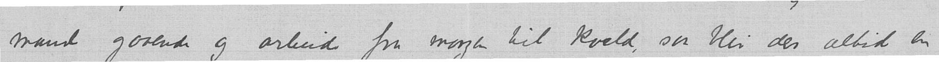mand gaaende og arbeide fra morgen til kveld, saa blir der altid en
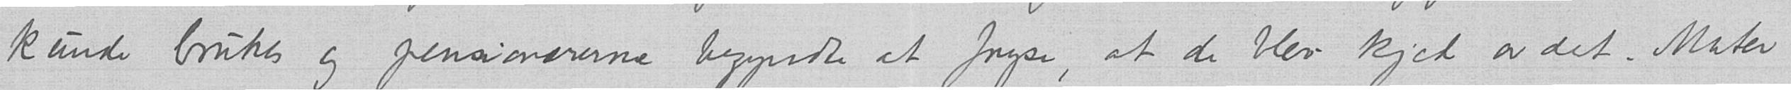kunde brukes og pensionærerne begyndte at fryse, at de blev kjed av det. Mater
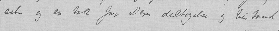selv og en tak for Deres deltagelse og bistand
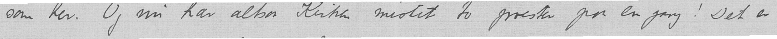som her. Og nu har altsaa Kirken mistet to prester paa en gang! Det er
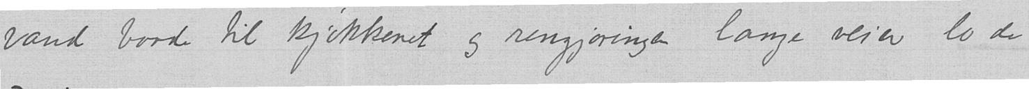vand baade til kjøkkenet og rengjøringen lange veier lo de
